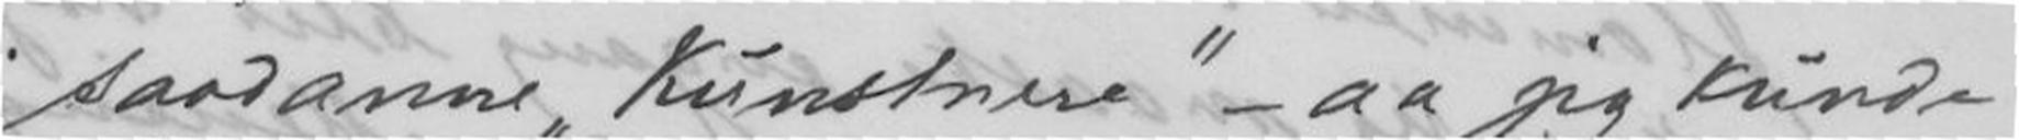saadanne Kunstnere - aa jeg kunde
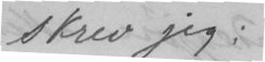skrev jeg:
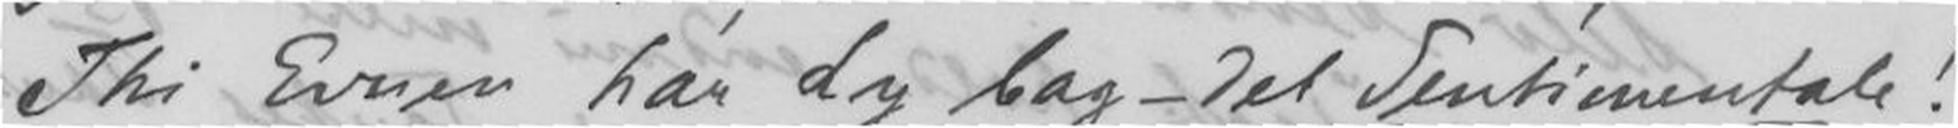Thi Evner har dy bag - det Sentimentale!
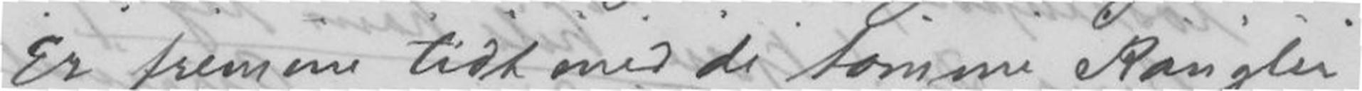Er fremme tidt med de tomme Rangler
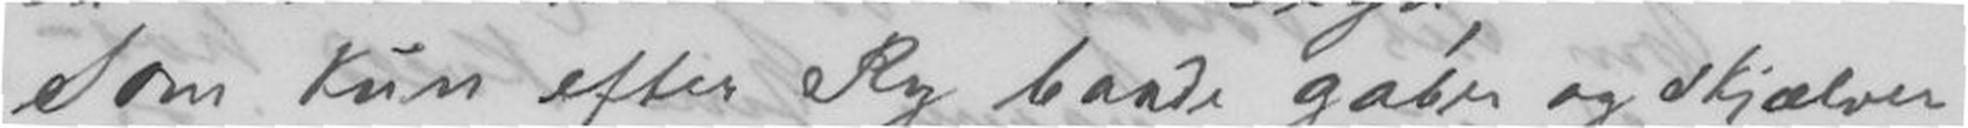som kun efter Ry baade gaber og skjælver
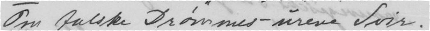Om falske Drømmes - urene Svir.
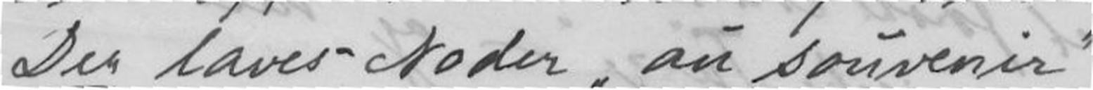Der laves - Noder au souvenir
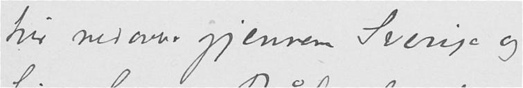tur nedover gjennem Sverige og
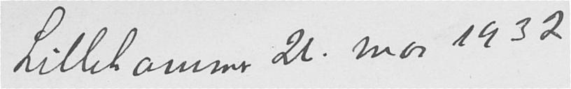Lillehammer 21. mai 1932
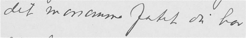det morsomme fatet du har
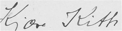Kjære Kitti,
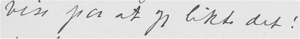viss paa at jeg likte det!
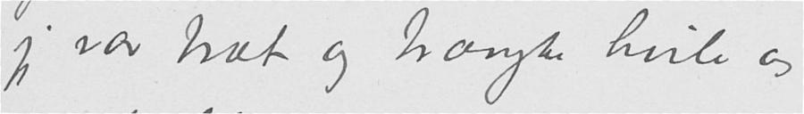jeg var træt og trængte hvile og
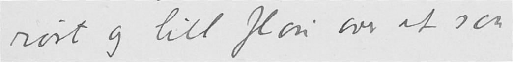rørt og litt flau over at saa
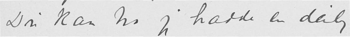Du kan tro jeg hadde en deilig
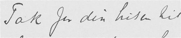Tak for din hilsen til
